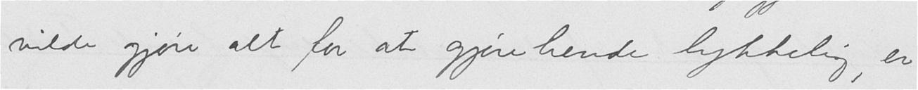vilde gjøre alt for at gjøre hende lykkelig, er
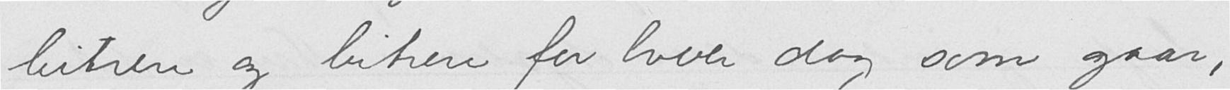bitrere og bitrere for hver dag som gaar,
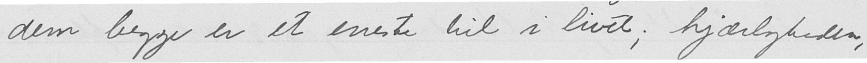dem begge er et eneste til i livet; kjærligheden,
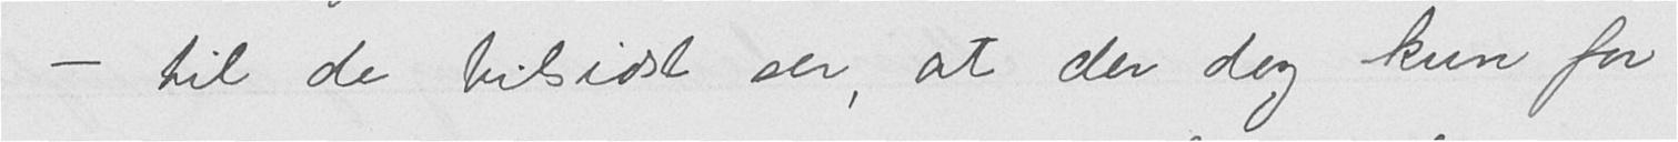- til de tilsidst ser, at der dog kun for
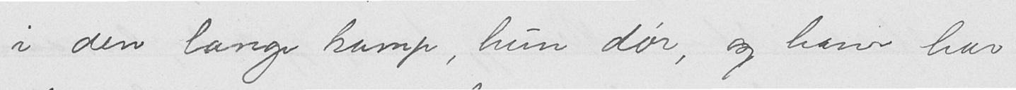i den lange kamp, hun dør, og ham har
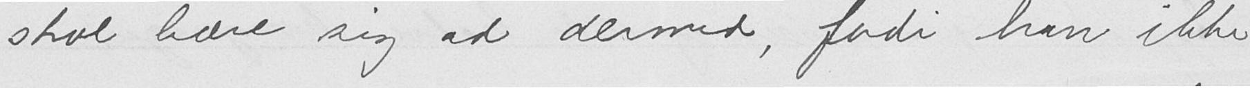skal bære sig ad dermed, fordi han ikke
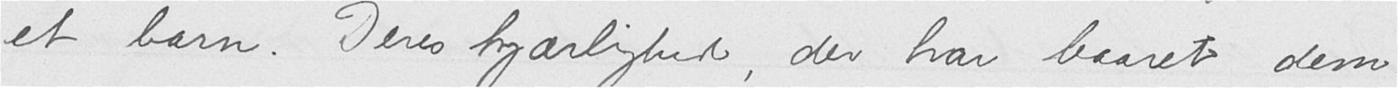et barn. Deres kjærlighed, der har baaret dem
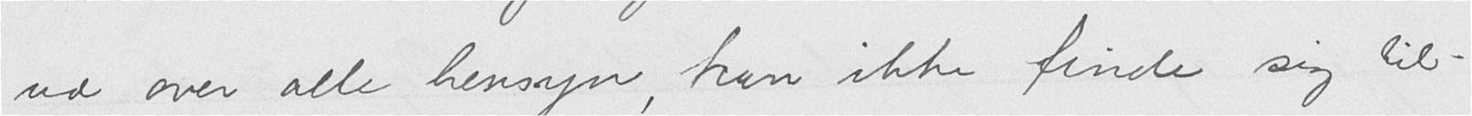ud over alle hensyn, kan ikke finde sig til-
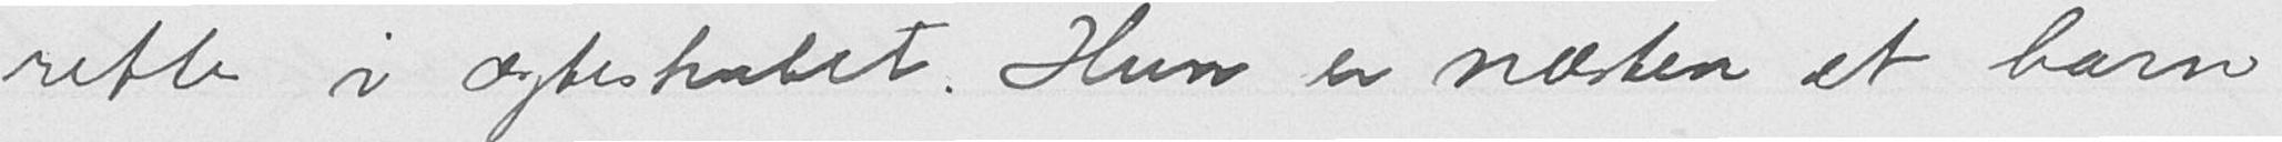rette i ægteskabet. Hun er næsten et barn
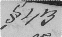§ 43
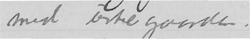med urtegaaarden.
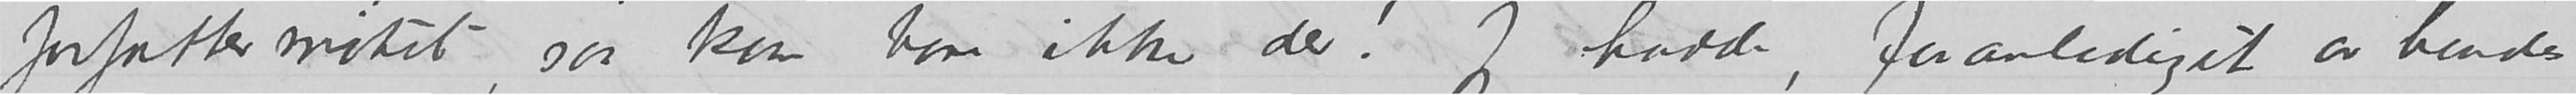forfattermøtet, saa kom hun bare ikke der!  Jeg hadde, foranlediget av hendes
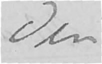Din
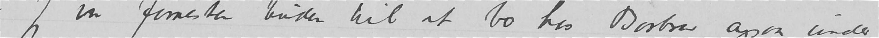– Jeg var forresten buden til at bo hos Barbra ogsaa under
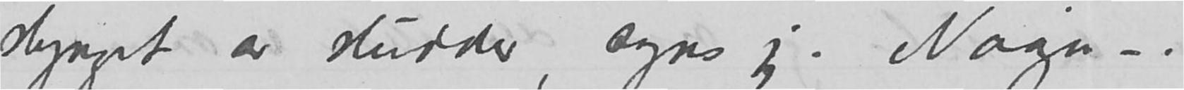slynget av sludder, synes jeg. Naaja –-.
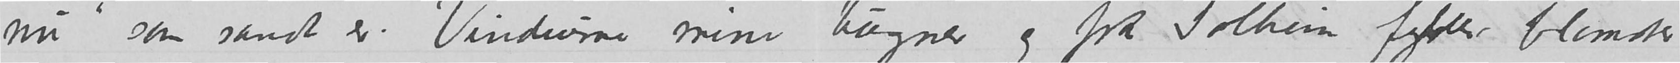nu» som sandt er. Vinduerne mine bugner og frk Solheim fylder blomster
